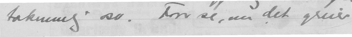taknemlig osv. Faar se, om det greier
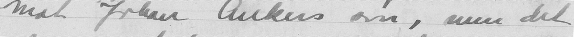mot Johan Ankers søn, men det
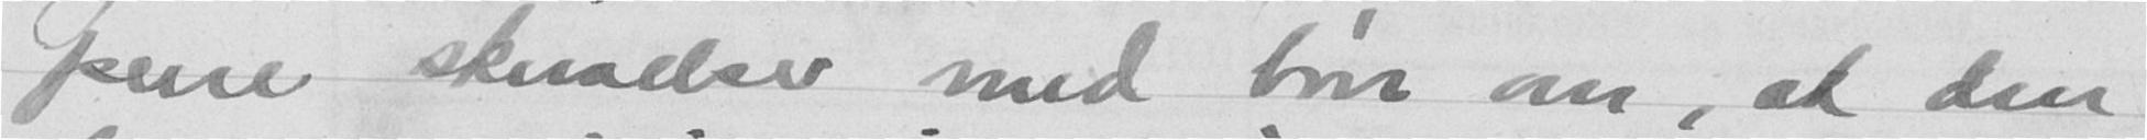pene skrivelser med bøn om, at den
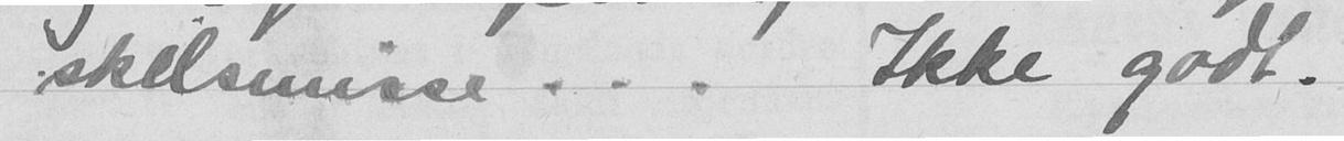skilsmisse... Ikke godt.
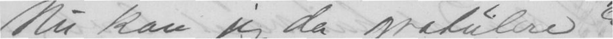Nu kan jeg da gratulære
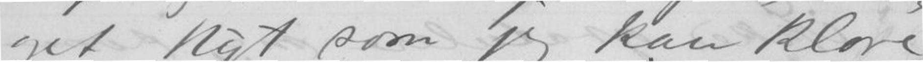get Nyt som jeg kan klore
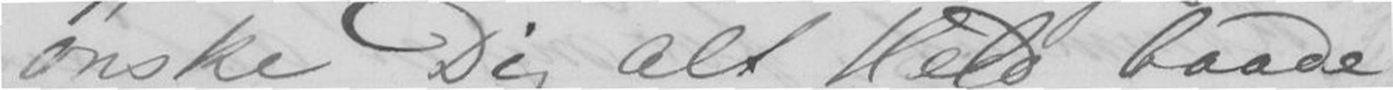ønske Dig alt Held baade
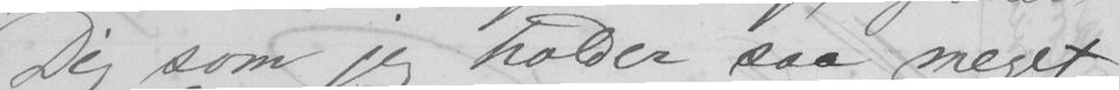Dig som jeg holder saa meget
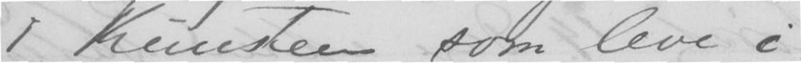i Kunsten som leve i
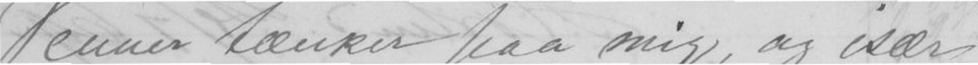Venner tænker paa mig, og især
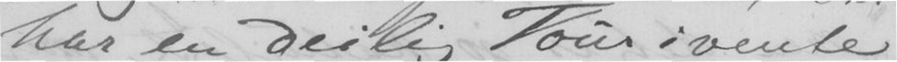har en deilig Tour i vente
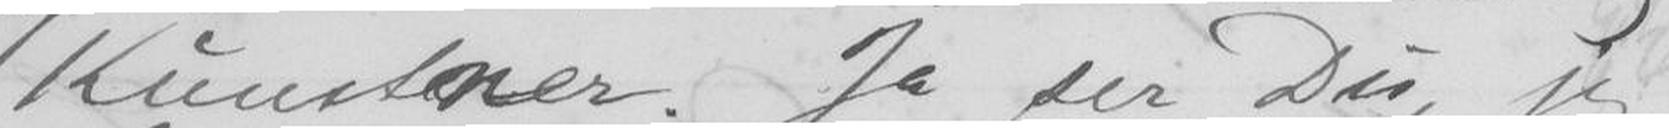Kunstner. Ja ser Du, jeg
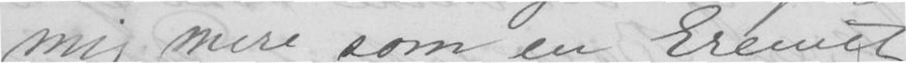mig mere som en Eremit;
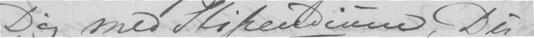Dig med Stipendium, Du
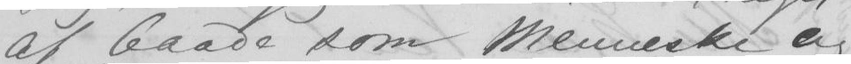af baade som Menneske og
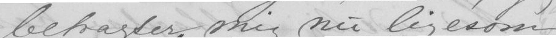betragter mig nu ligesom
